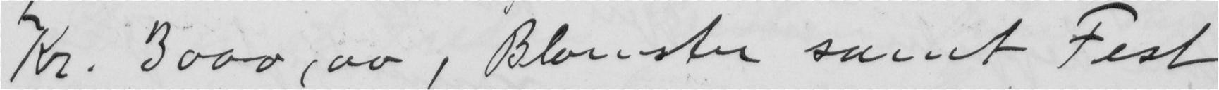Kr. 3000, av, Blomster samt Fest
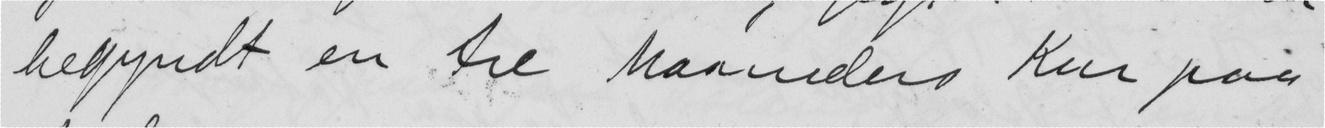begyndt en tre Maaneders Kur paa
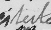citerte
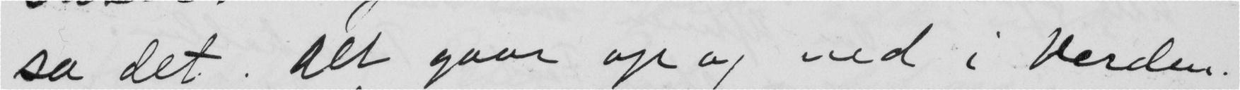sa det. Alt gaar op og ned i Verden.
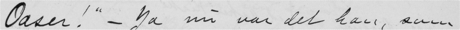Oaser!" — Ja nu var det han, som
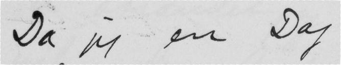Da jeg en Dag
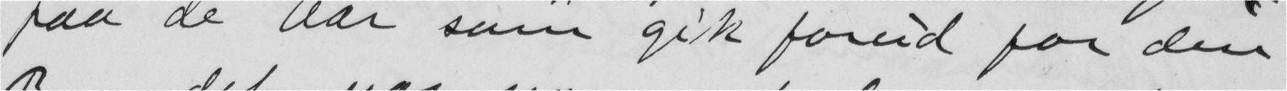paa de Aar som gik forud for den
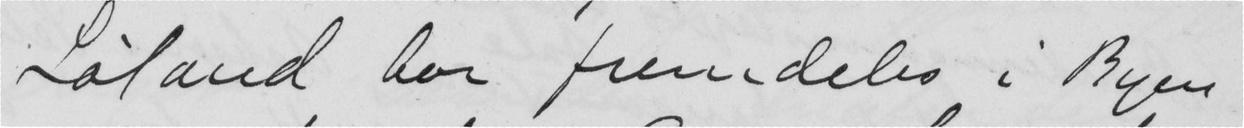Løland bor fremdeles i Byen
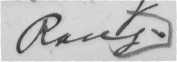Rang.
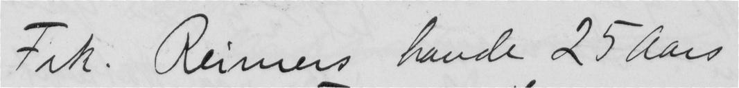Frk. Reimers havde 25 Aars
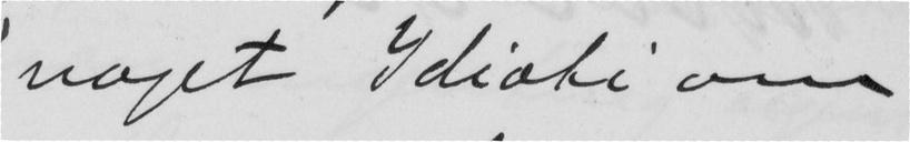noget Idioti om
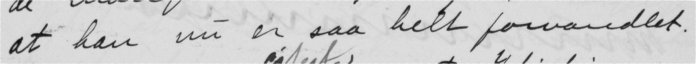at han nu er saa helt forvandlet.
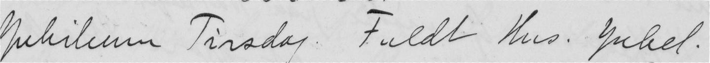Jubileum Tirsdag. Fuldt Hus. Jubel.
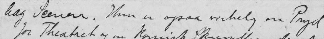bag Scenen. Hun er ogsaa virkelig en Pryd
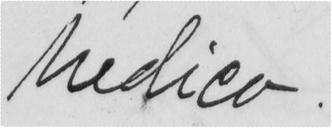Medico.
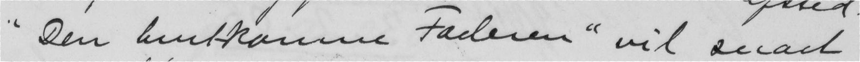"Den bortkomne Faderen" vil snart
